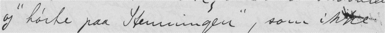og "hørte paa Stemningen", som ikke
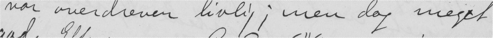var overdreven livlig; men dog meget
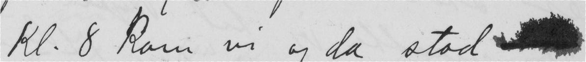Kl. 8 kom vi og da stod
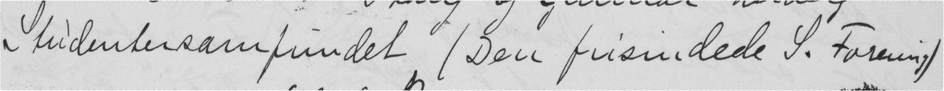Studentersamfundet (Den frisindede S. Forening)
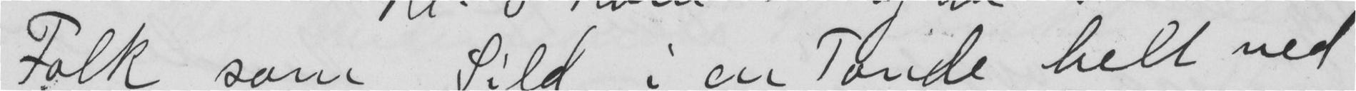Folk som Sild i en Tønde helt ned
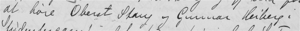at høre Oberet Stang og Gunnar Heiberg i
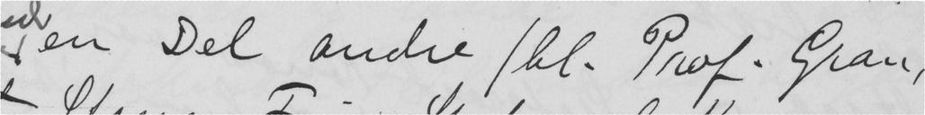en Del andre (bl. Prof. Gran,
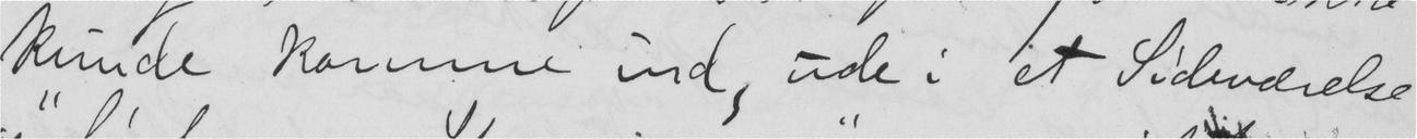kunde komme ind, ude i et Sideværelse
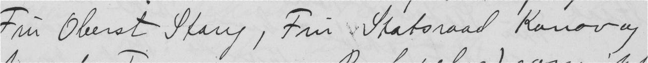Fru Oberst Staug, Fru Statsraad Konov og
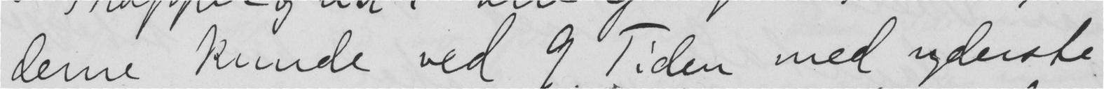derne kunde ved 9 Tiden med yderste
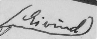Eivind
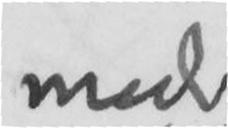med
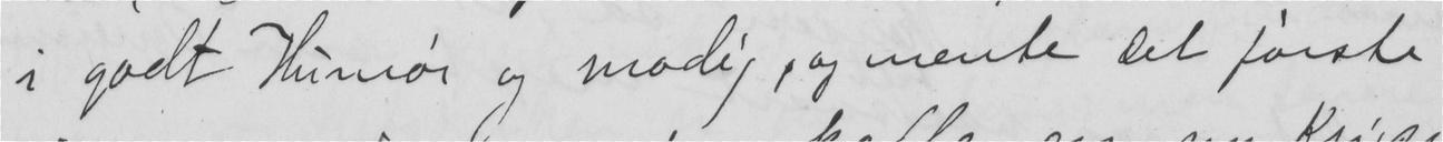i godt Humør og modig, og mente det første
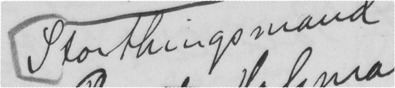Stortningsmand
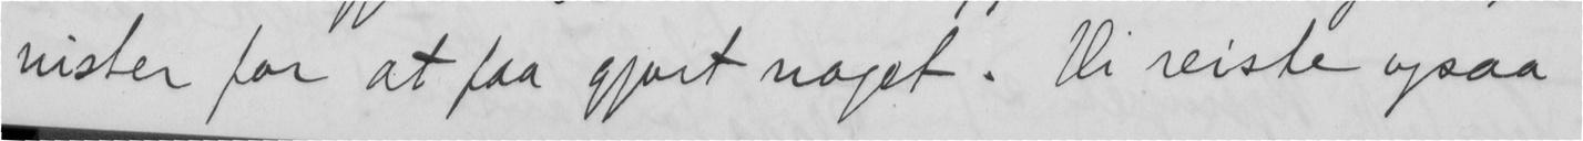nister for at faa gjort noget. Vi reiste ogsaa
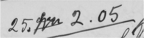25. 2.05
